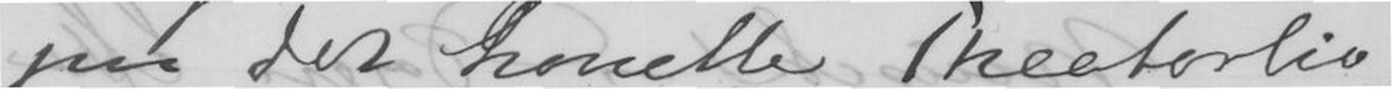paa det honette Theaterliv.
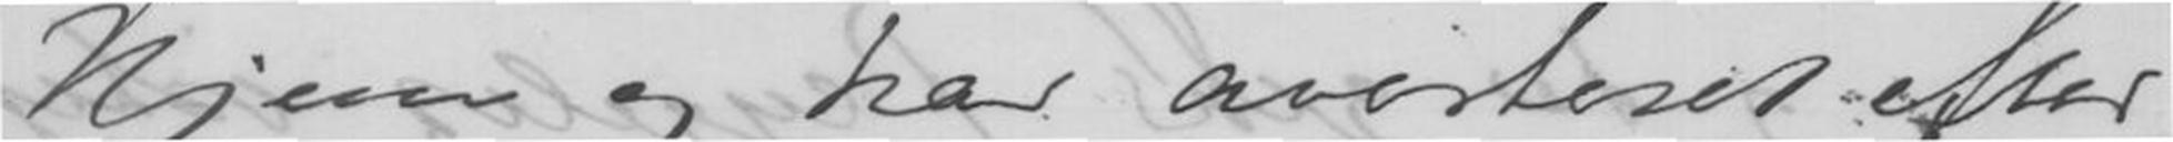Hjem og har averteret efter
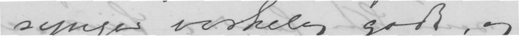synger virkelig godt, og
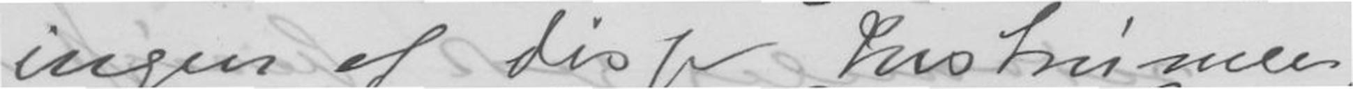ingen af disse Instrumen
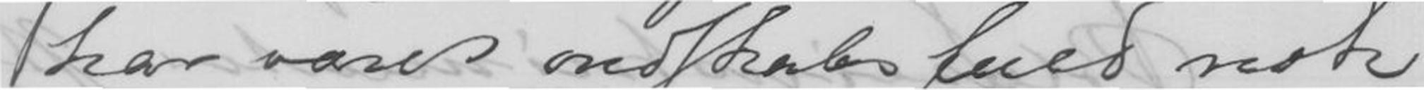har være ondskabsfuld nok
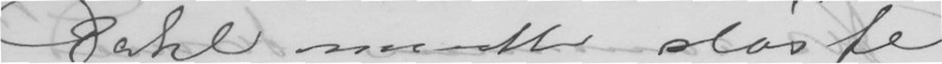Dahl maatte sløife
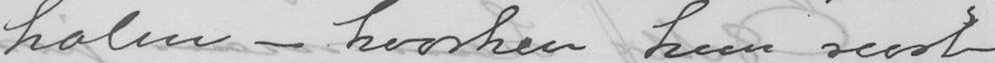holm - hvorhen hun reiste
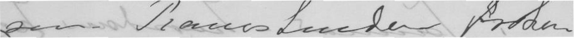saa Pianistinden, frøken
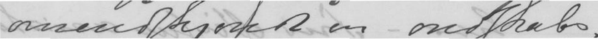omendskjøndt en ondskabs-
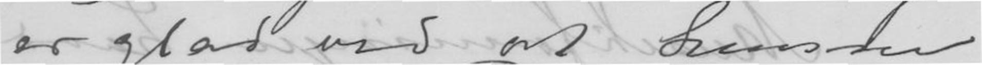er glad ved at kunne
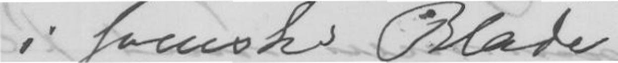i svenske Blade
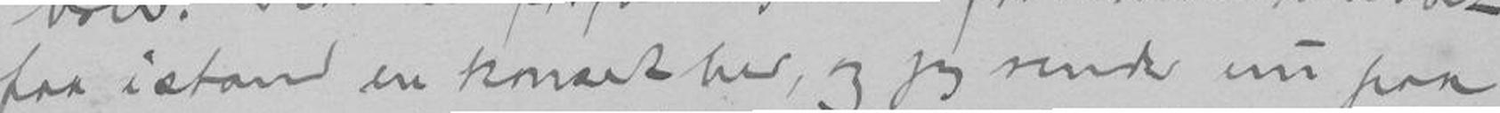faa istand en konsert her, og jeg venter nu paa
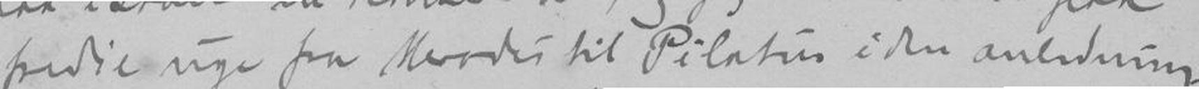tredie uge fra Herodes til Pilatus i den anledning,
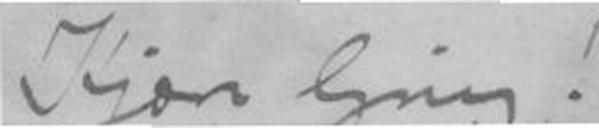Kjære Grieg!
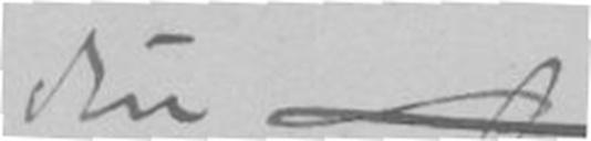Din
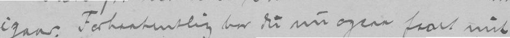igaar. Forhaabentlig har du nu ogsaa faaet mit
